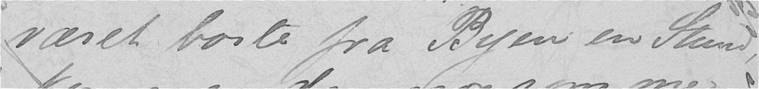været borte fra Byen en Stund,
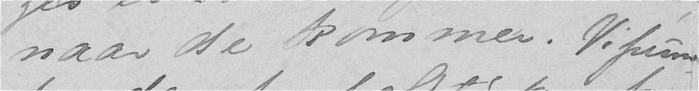naar de kommer. Vi pum-
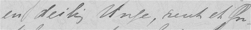en deilig Unge, rent et Øn-
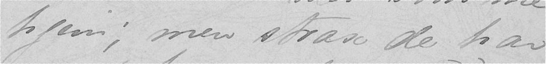hjem; men strax de har
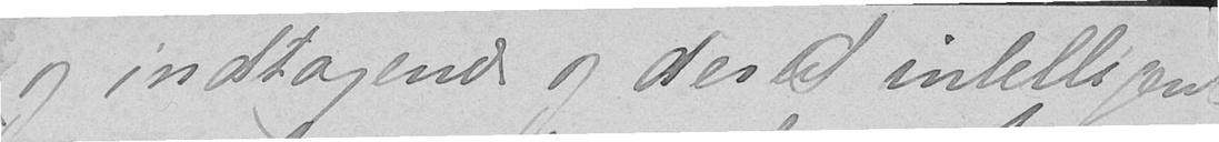og indtagende og dertil intelligent
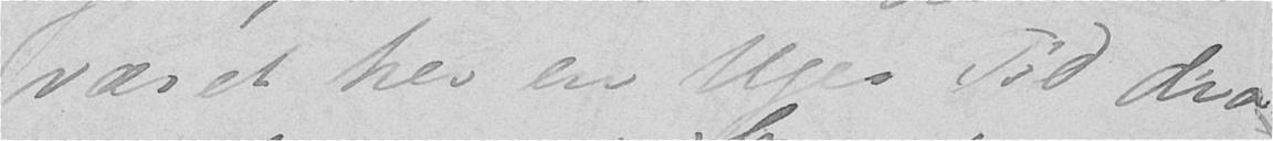været her en Uges Tid dra-
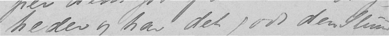heder og har det godt den Stund
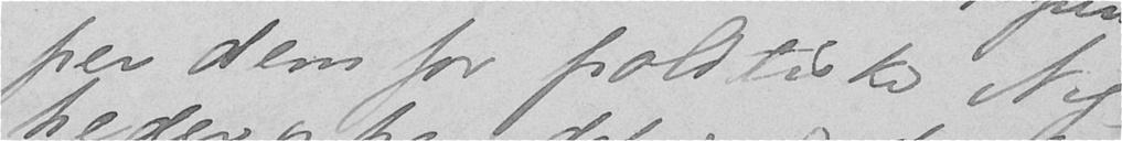per dem for politiske Ny-
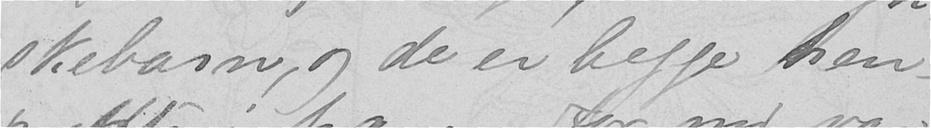skebarn, og de er begge hen-
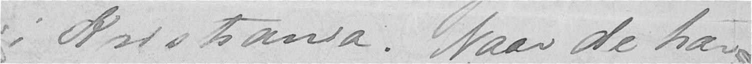i Kristiana. Naar de har
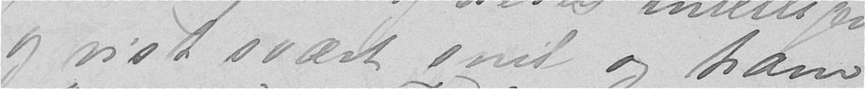og vist svært snil og ham
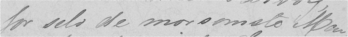for selv de morsomste Men-
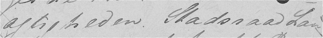agtigheden. Stadsraad Lan-
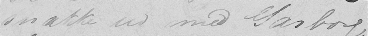snakke ud med Garborg,
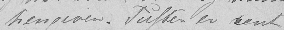hengiven. Tuften er rent
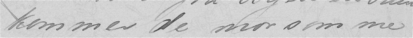kommer de morsomme
